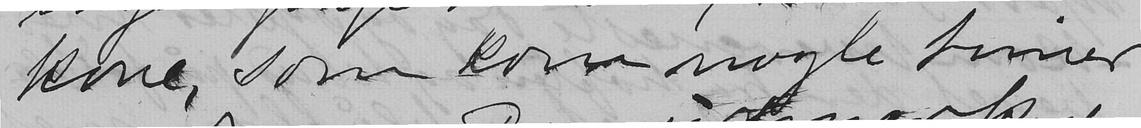kone, som kom nogle timer
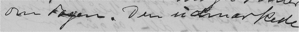om dagen. Den udmærkede
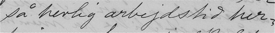så herlig arbejdstid her-
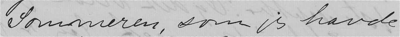Sommeren, som jeg havde
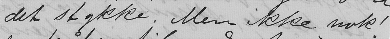det stykke. Men ikke nok!
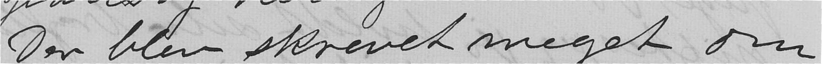Der blev skrevet meget om
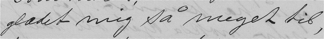glædet mig så meget til,
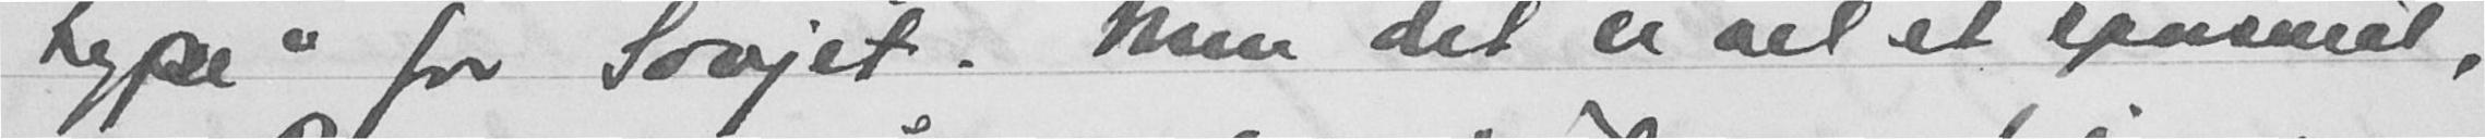type" for Sovjet. Men det er vel et spørsmål,
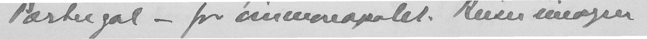Portergal - for vinmonopolet. Reiser imorgen.
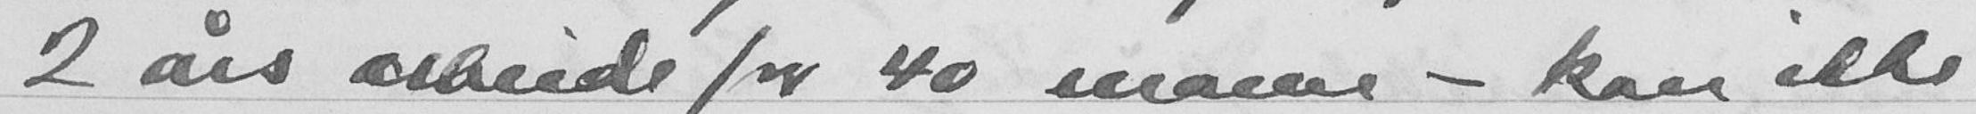2 års arbeide for 40 mann - kan ikke
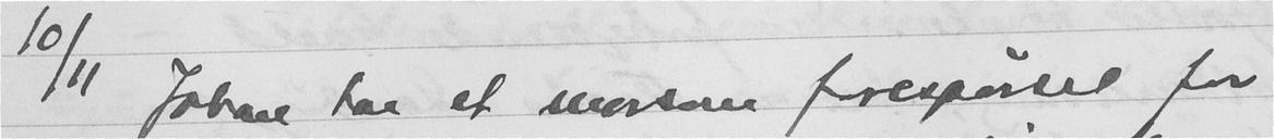15/11 Johan har et morsom forespørsel for
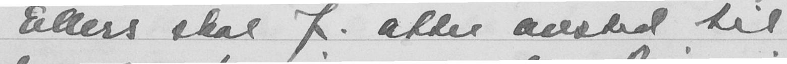Ellers skal J. atter avsted til
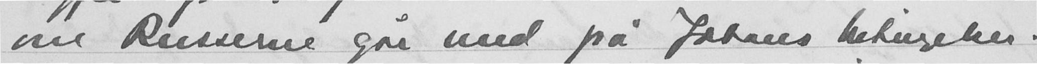om Resserne går med på Johans betingelser.
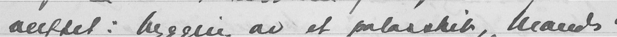verftet i bygging av et polarskib, "Mauds-
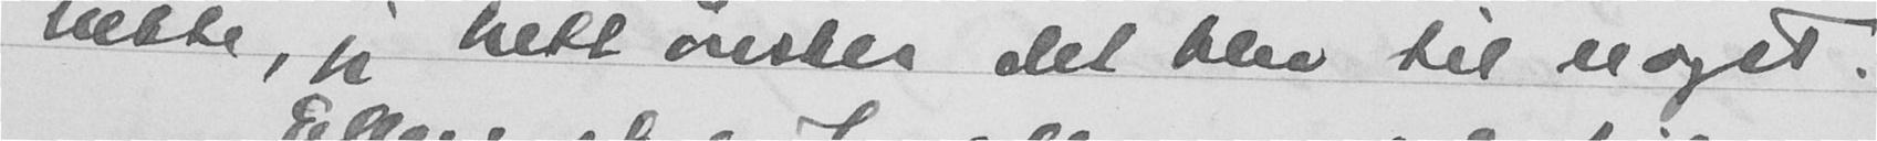nekte, jeg hett ønsker det blir til noget.
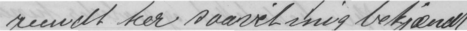rundt her saavit mig bekjændt
